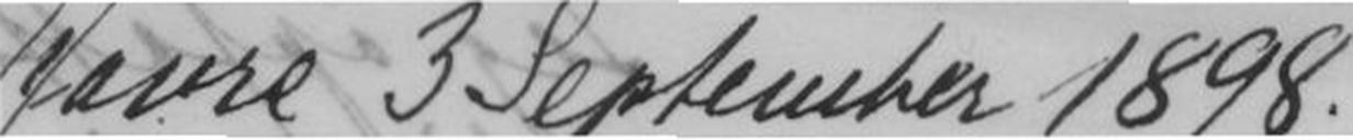Havre 3 September 1898.
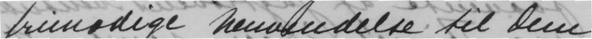frimodige henvendelse til Dem
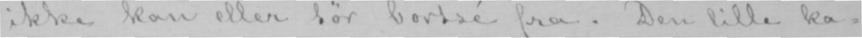ikke kan eller tør bortsé fra. Den lille ka-
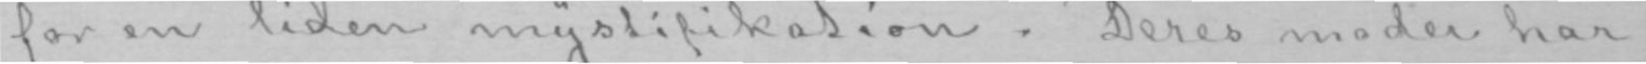for en liden mystifikation. Deres moder har
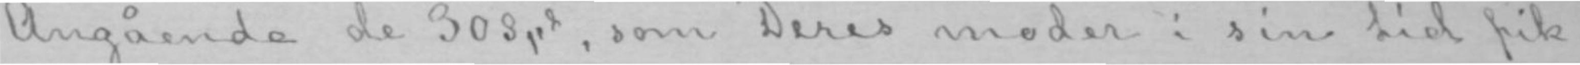Angående de 30 Spd, som Deres moder i sin tid fik
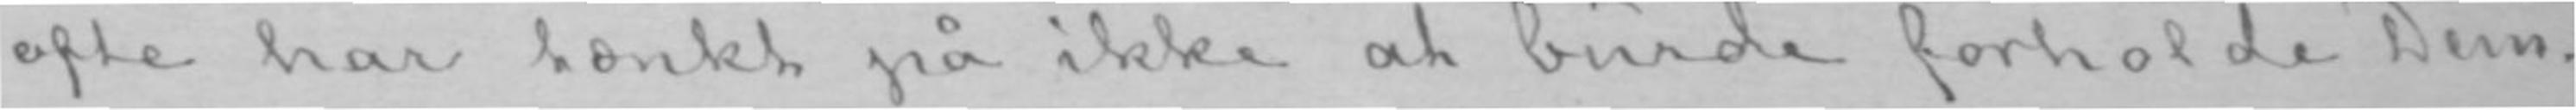ofte har tænkt på ikke at burde forholde Dem.
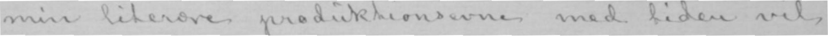min literære produktionsevne med tiden vil
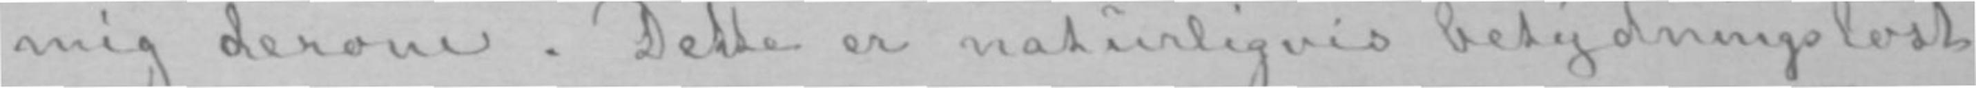mig derom. Dette er naturligvis betydningsløst
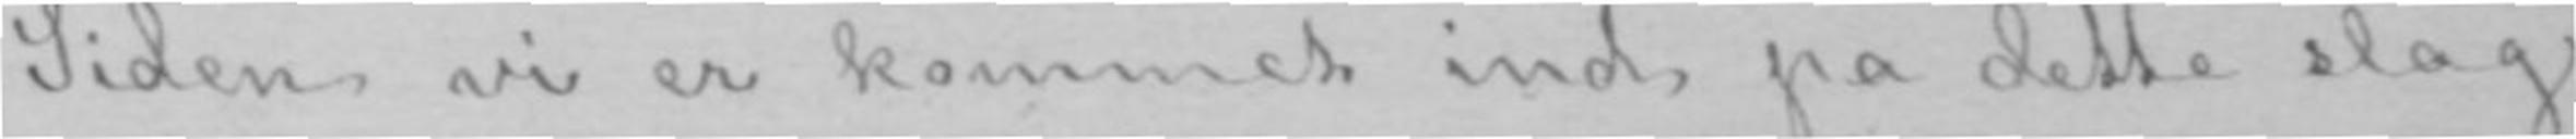Siden vi er kommet ind på dette slags
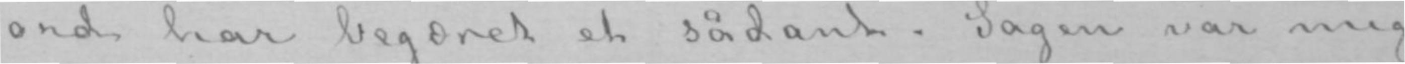ord har begæret et sådant. Sagen var mig
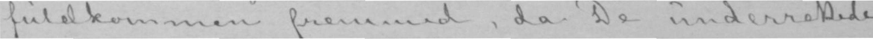fuldkommen fremmed, da De underrettede
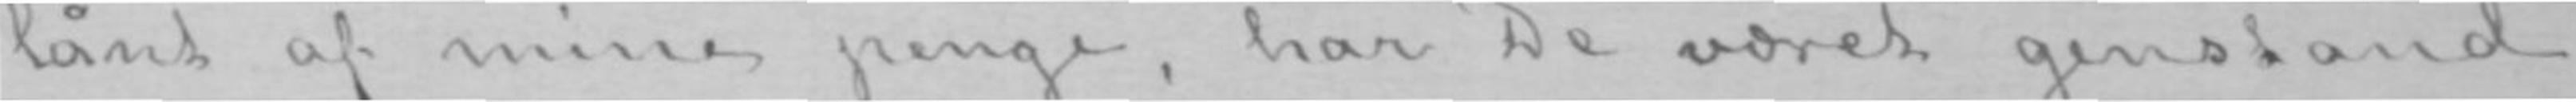lånt af mine penge, har De været genstand
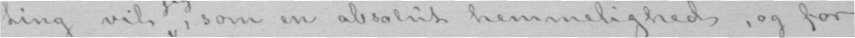ting vil jeg, som en absolut hemmelighed, og for
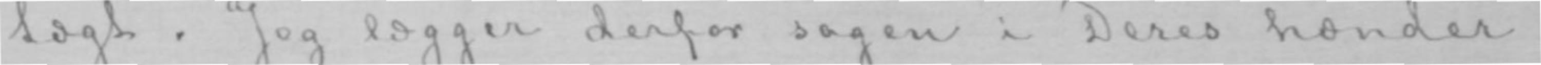tægt. Jeg lægger derfor sagen i Deres hænder,
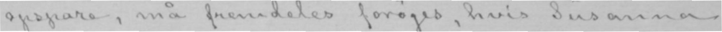opspare, må fremdeles forøges, hvis Susanna
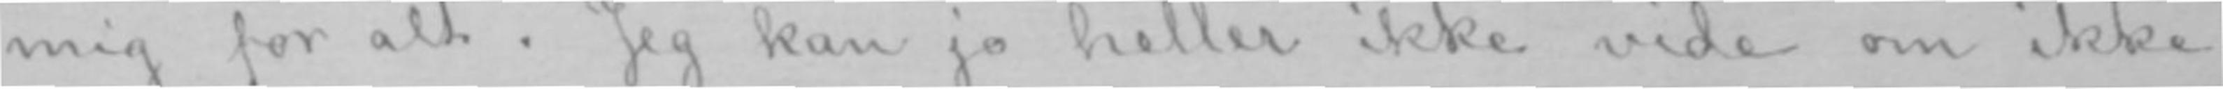mig for alt. Jeg kan jo heller ikke vide om ikke
